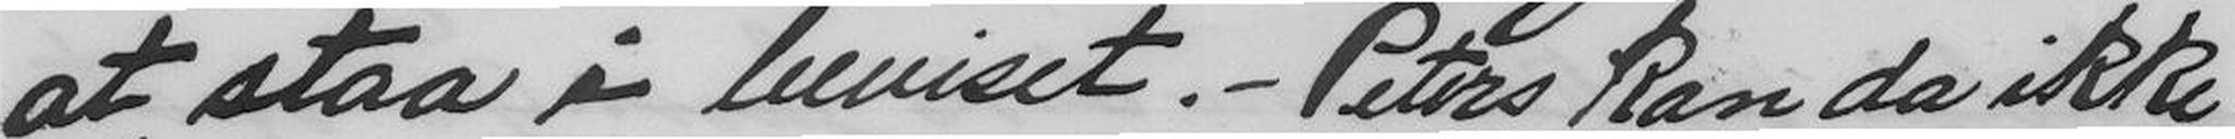at staa i beviset. - Peters kan da ikke
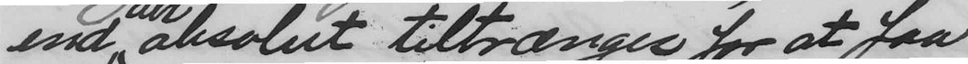end det absolut tiltrænges for at faa
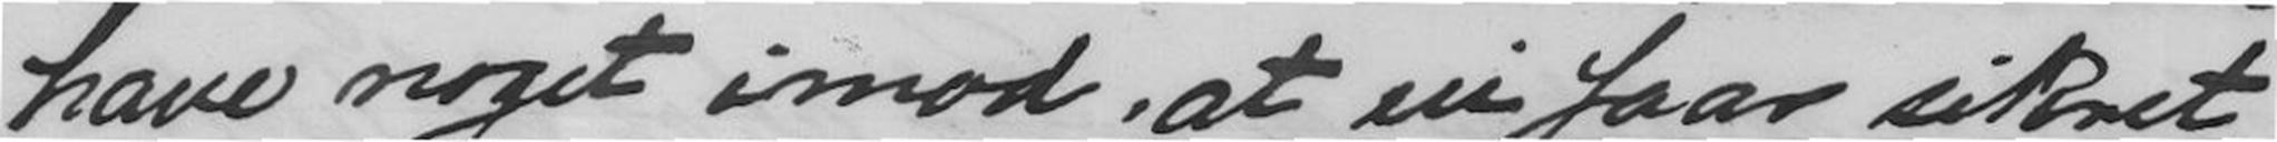have noget imod, at vi faar sikret
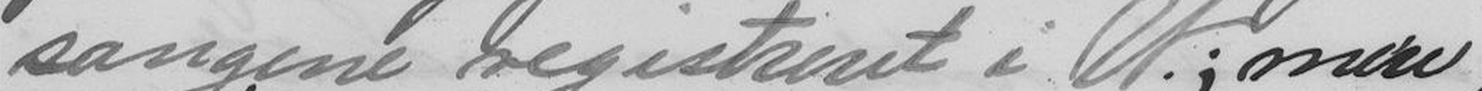sangene registreret i W.; men
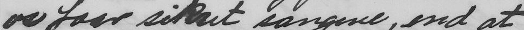os faar sikret sangene, end at
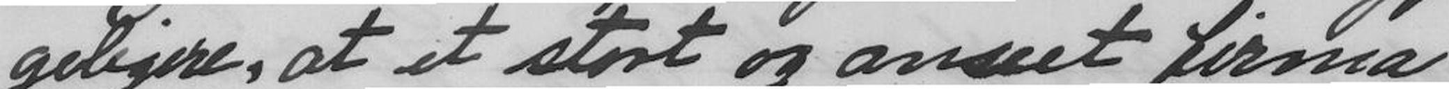geligere, at et stort og anseet firma
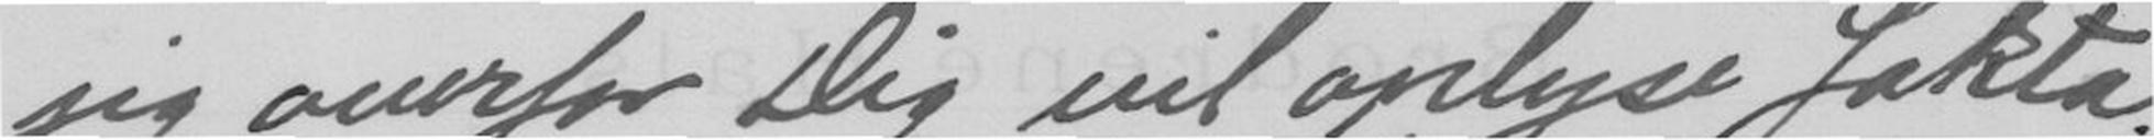jeg overfor Dig vil oplyse fakta.
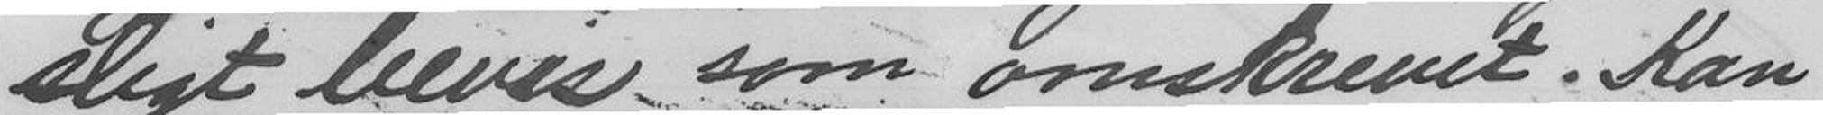sligt bevis som omskrevet. Kan
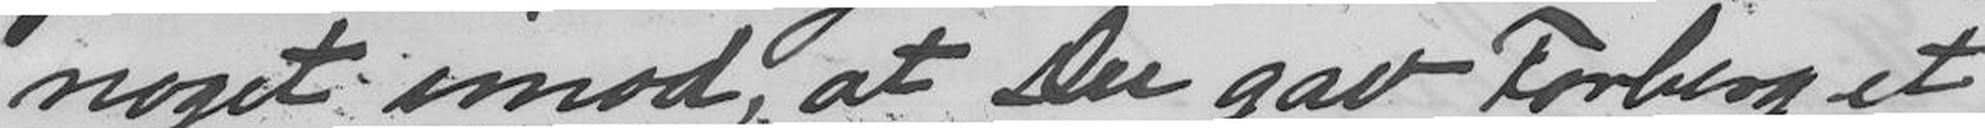noget imod, at Du gav Forberg et
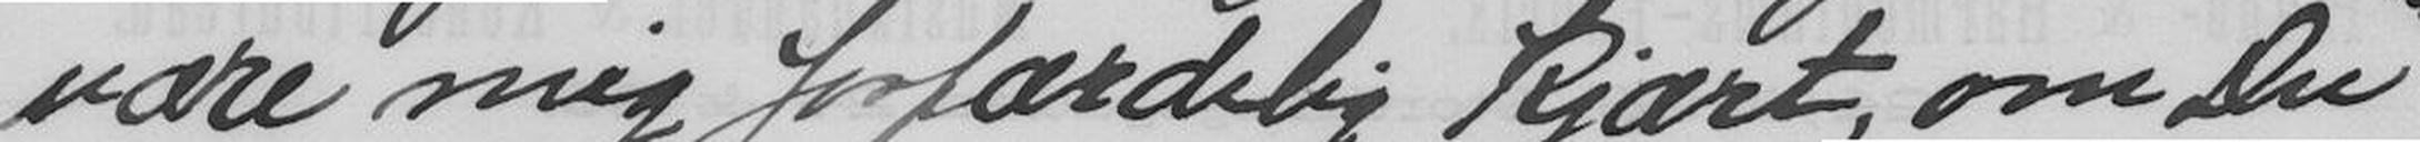være mig forfærdelig kjært, om Du
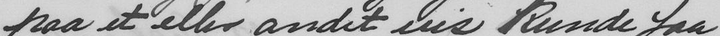paa et eller andet vis kunde faa
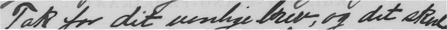Tak for dit venlige brev, og det skulde
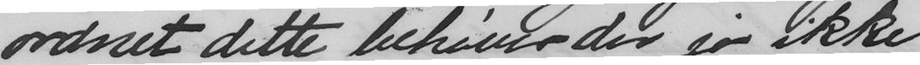ordnet dette behøver der jo ikke
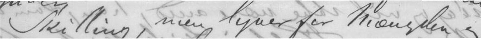Skilling, men lyver for Mængden og
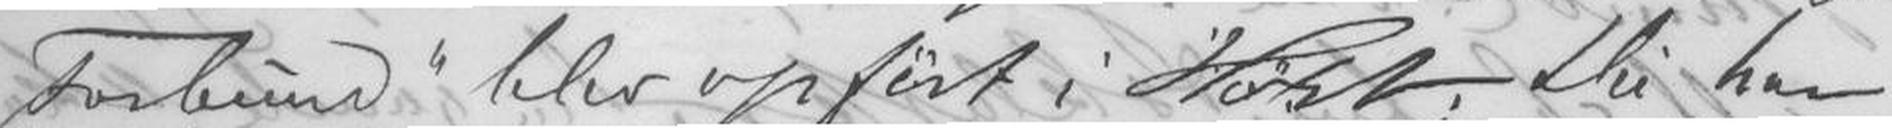Forbund blev opført i Høst; Du har
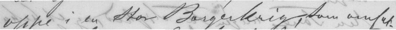oppe i en stor Borgerkrig, som omfat-
